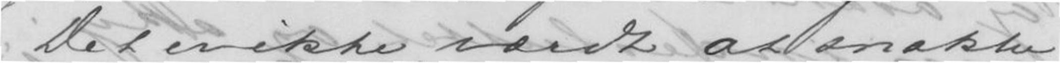Det er ikke værdt at snakke
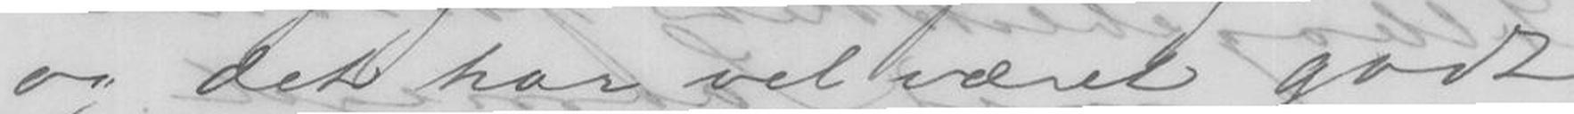og det har vel været godt
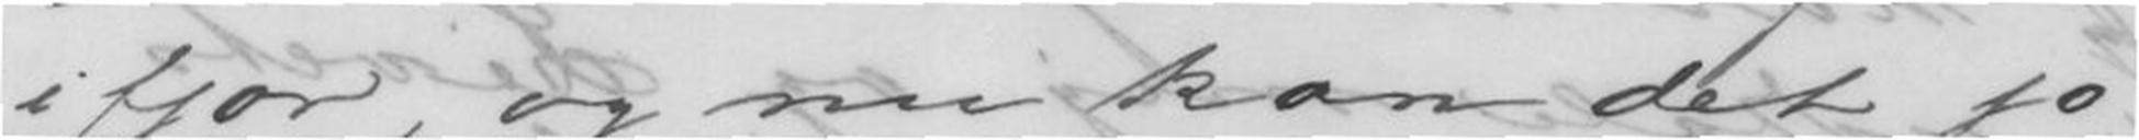ifjor, og nu kan det jo
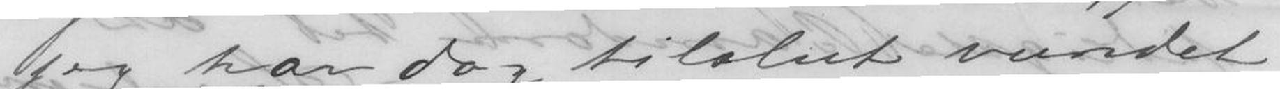for jeg har dog tilslut vundet
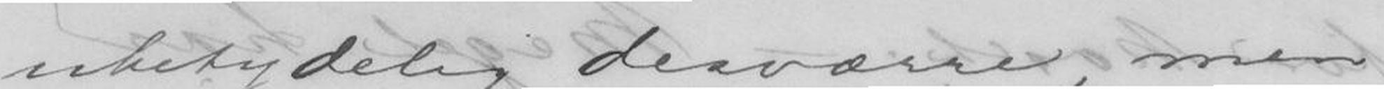ubetydelig desværre, men
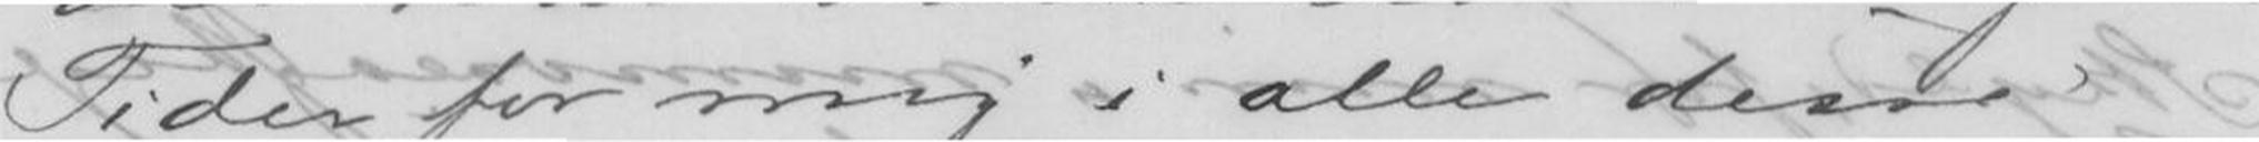Tider for mig i alle disse
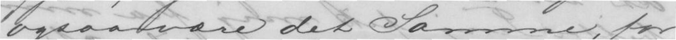ogsaa være det samme,
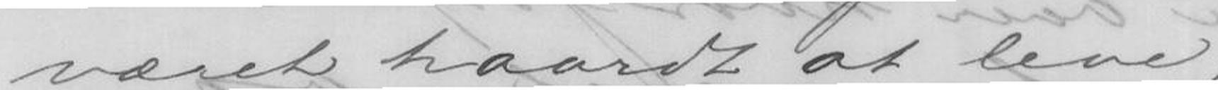været haardt at leve,
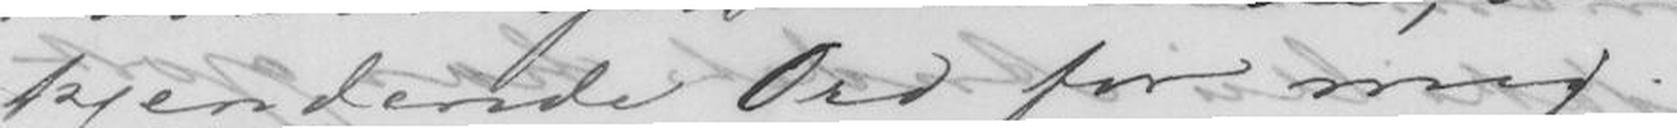kjendende Ord for mig.
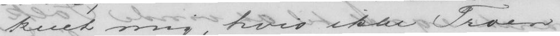kuet mig, hvis ikke Troen
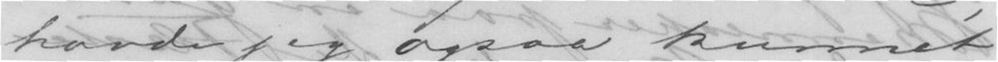havde jeg ogsaa kunnet
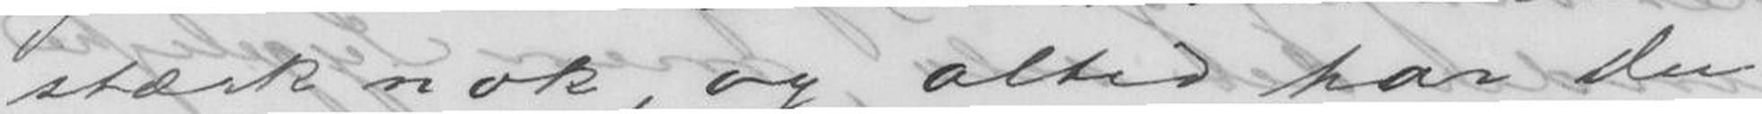stærk nok, og altid har Du
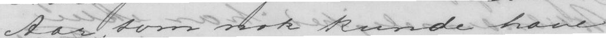Aar, som nok kunde have
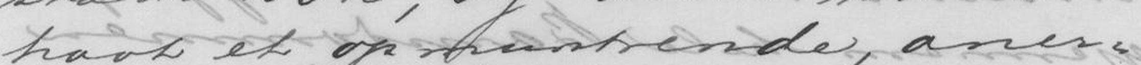havt et opmuntrende, aner-
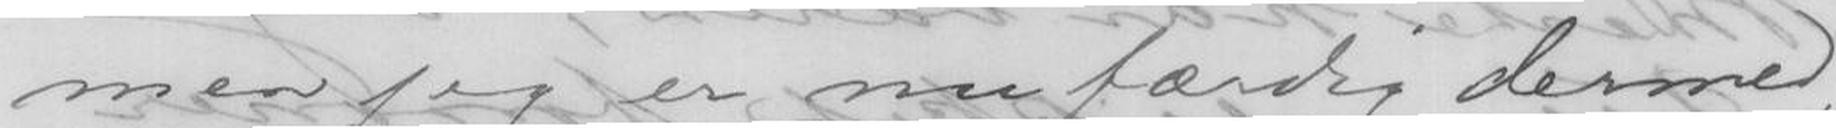men jeg er nu færdig dermed,
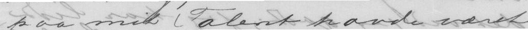paa mit Talent havde været
